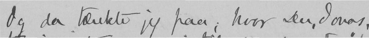Og da tænkte jeg paa; hvor Du, Jonas,
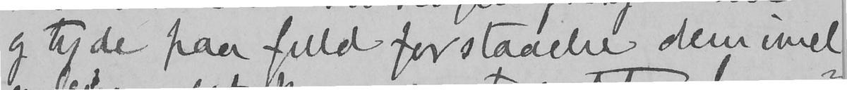og tyde paa fuld forstaaelse dem imel-
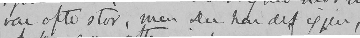var ofte stor, men Du har det igjen,
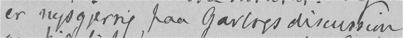er nysgjerrig paa Garborgs discussion
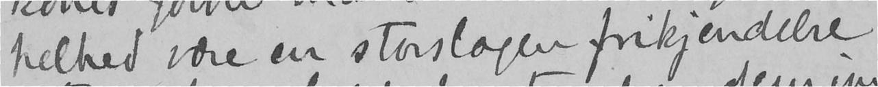helhed være en storslagen frikjendelse
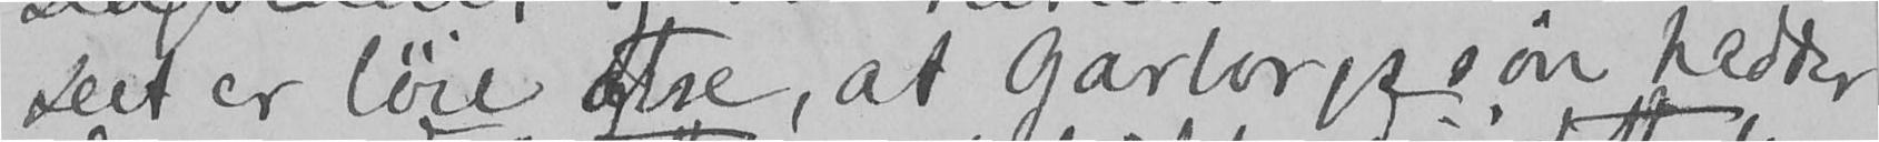Det er løie at se, at Garborgs søn hedder
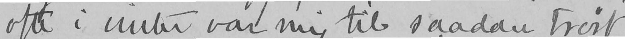ofte i vinter var mig til saadan trøst,
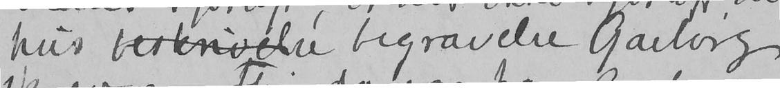hus beskrivelse begravelse Garborg
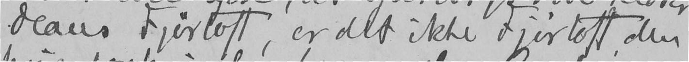Olaus Fjørtoft, er det ikke Fjørtoft, den
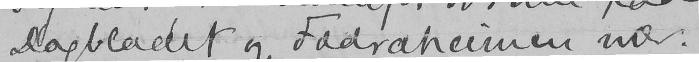Dagbladet og Fædraheimen nær.
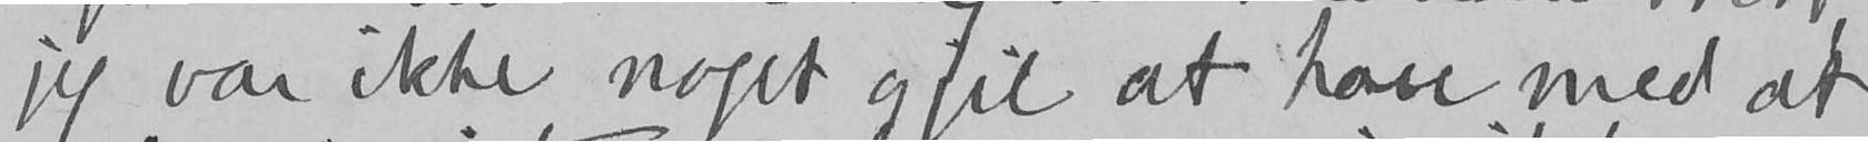jeg var ikke noget gjil at have med at
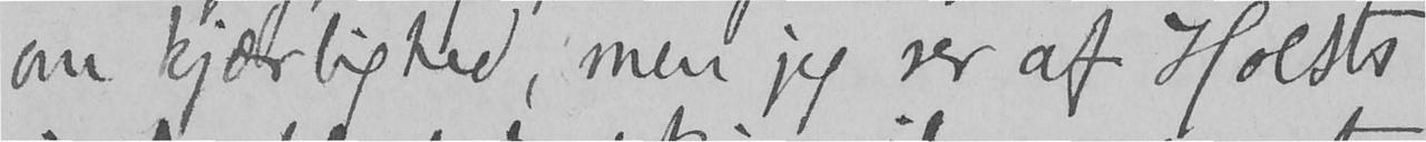om kjærlighed, men jeg ser af Holsts
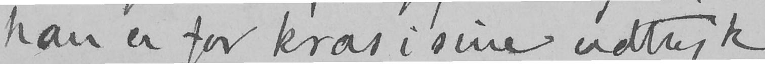han er for kras i sine udtryk.
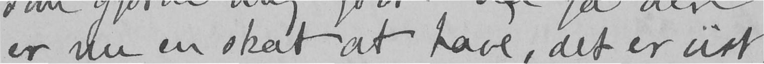er nu en skat at have, det er vist.
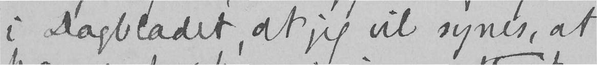i Dagbladet, at jeg vil synes, at
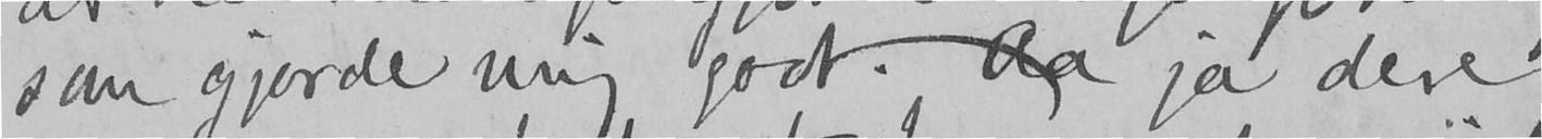som gjorde mig godt. Aa ja dere
